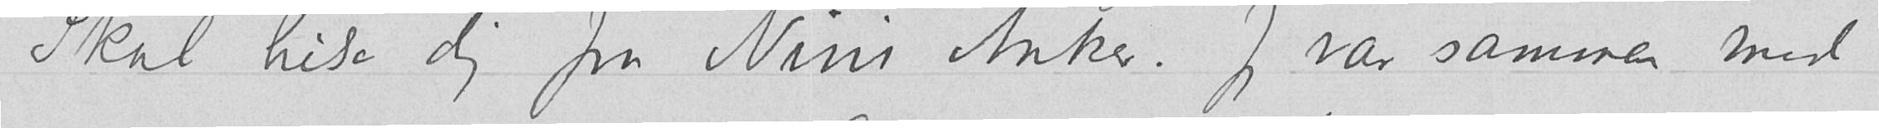Skal hilse dig fra Nini Anker. Jeg var sammen med
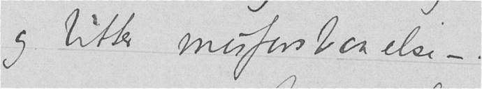og bitter misforstaaelse –.
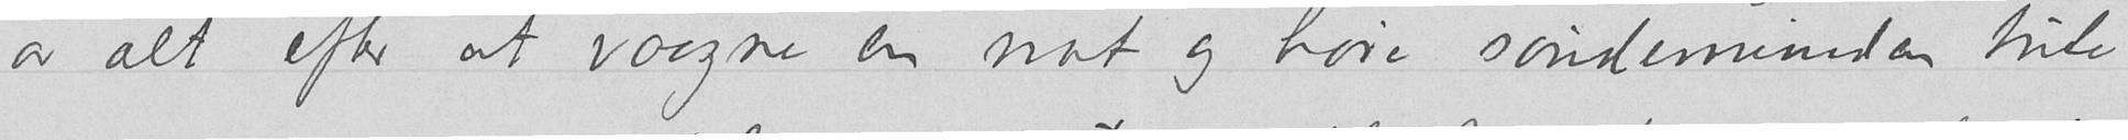av alt efter at vaagne en nat og høre søndenvinden tute
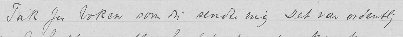Tak for boken som du sendte mig. Det var ordentlig
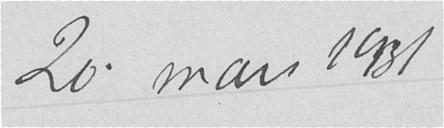20. mars 1931
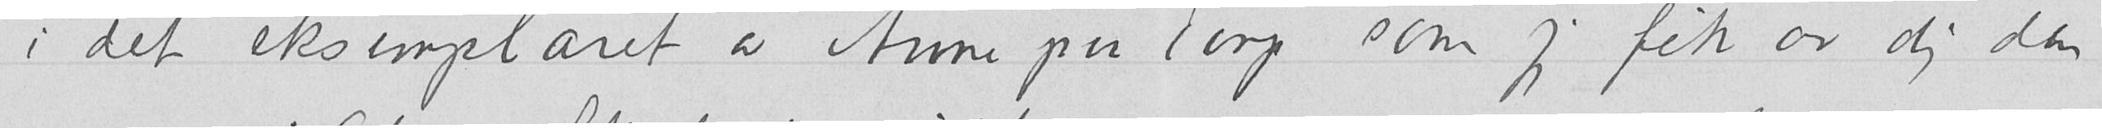i det eksemplaret av Anne paa Torp som jeg fik av dig den
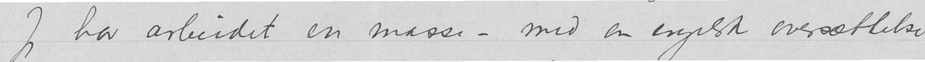Jeg har arbeidet en masse – med en engelsk oversættelse
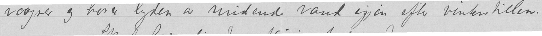vaagner og hører lyden av rindende vand igjen efter vinterstillen.
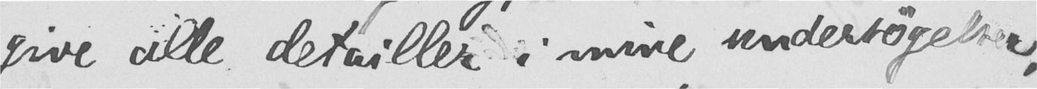give alle detailler i mine undersøgelser,
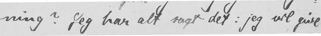ning? Jeg har alt sagt det: jeg vil give
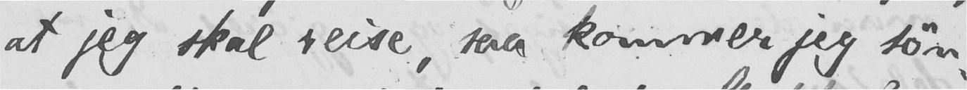at jeg skal reise, saa kommer jeg søn-
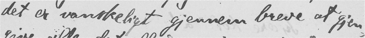det er vanskeligt gjennem breve at gjen-
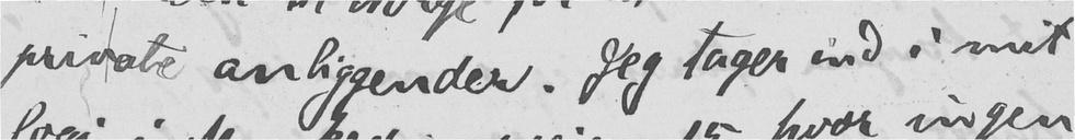private anliggender. Jeg tager ind i mit
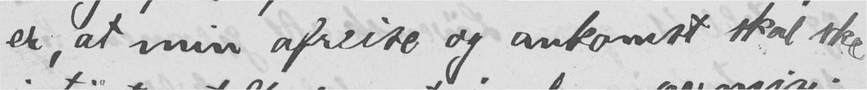er, at min afreise og ankomst skal ske
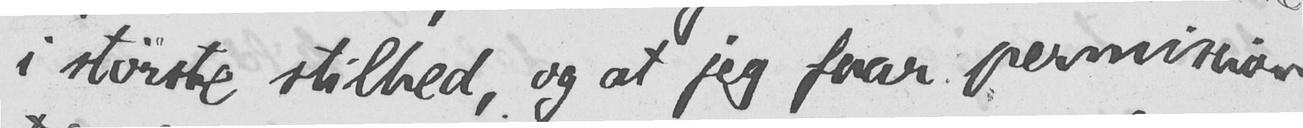i største stilhed, og at jeg faar permission
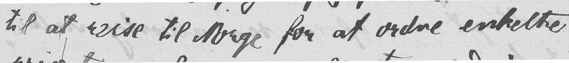til at reise til Norge for at ordne enkelte
